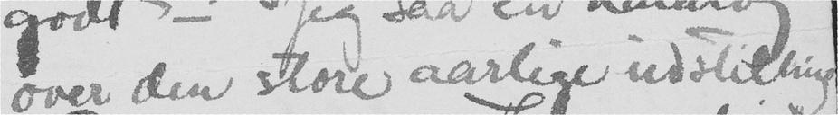over den store aarlige udstilling
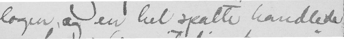logen, og en hel spalte handlede
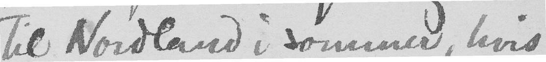til Nordland i sommer, hvis
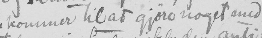kommer til at gjøre noget med
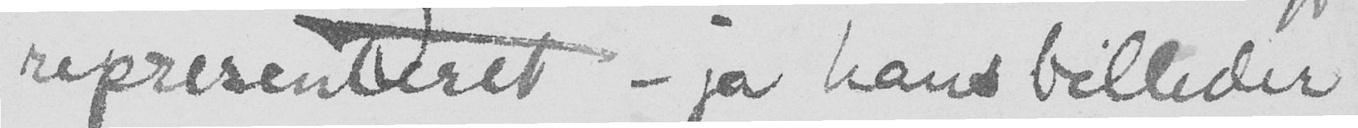representeret - ja hans billeder
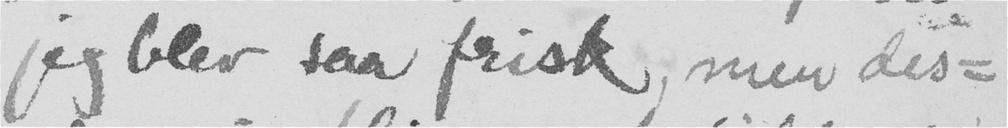jeg blev saa frisk; men des-
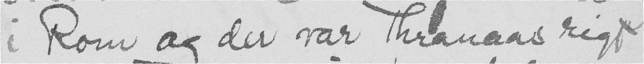i Rom og der var Thranaas rigt
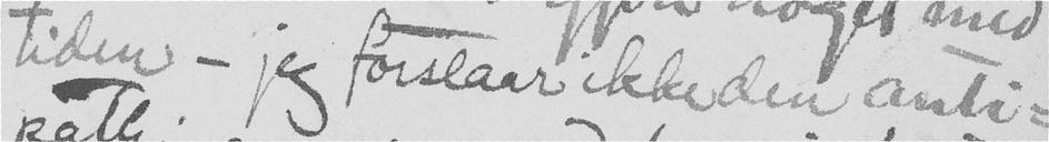tiden - jeg forstaar ikke den anti-
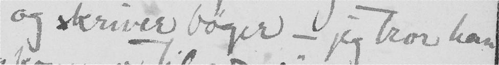og skriver bøger - jeg tror han
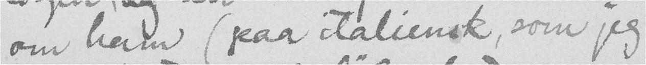om ham (paa italiensk, som jeg
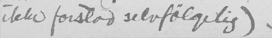ikke forstod selvfølgelig).
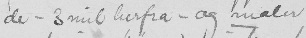de - 3mil herfra - og maler
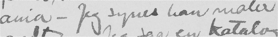ania. - Jeg synes han maler
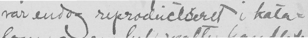var endog reproducteret i kata-
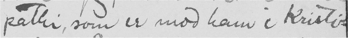pathi, som er mod ham i Kristi-
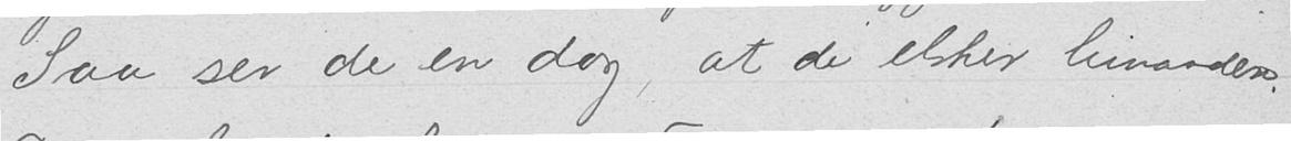Saa ser de en dag, at de elsker hinanden.
